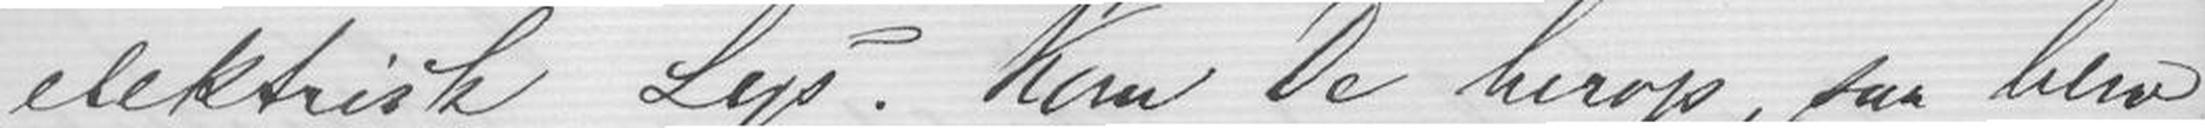alektrisk Lys. Kom De herop, saa blev
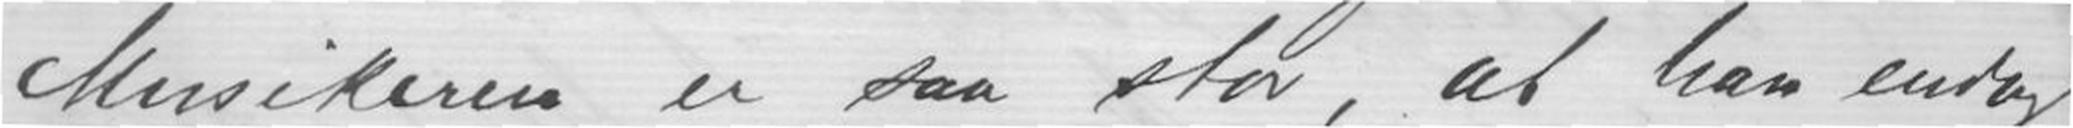Musikeren er saa stor, at han endog
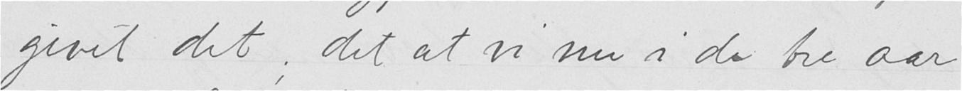givet det; det at vi nu i de tre aar
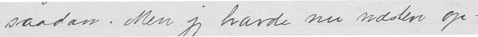saadan. Men jeg havde nu næsten op-
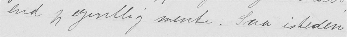end jeg egentlig mente. Saa isteden
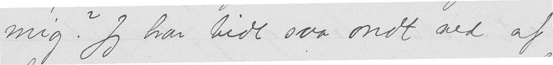mig? Jeg har lidt saa ondt ved at
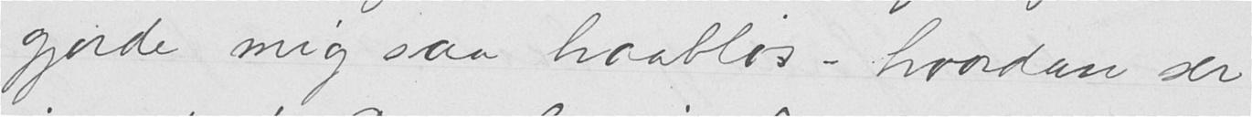gjorde mig saa haabløs - hvordan ser
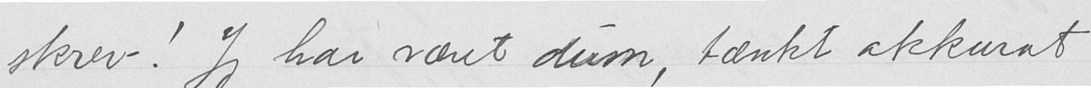skrev! Jeg har været dum, tænkt akkurat
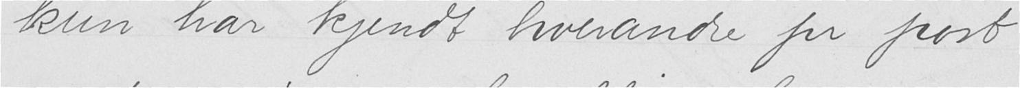kun har kjendt hverandre pr post
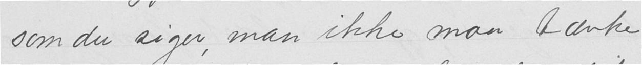som du siger, man ikke maa tænke -
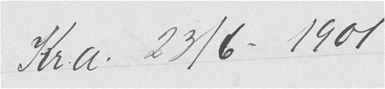Kr.a. 23/6-1901
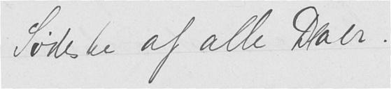Sødeste af alle Deaer!
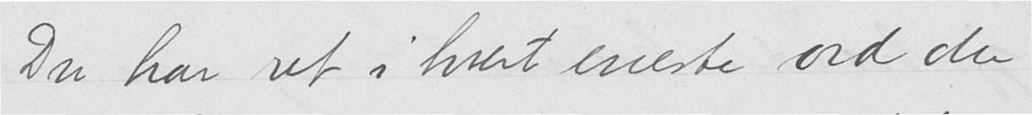Du har ret i hvert eneste ord du
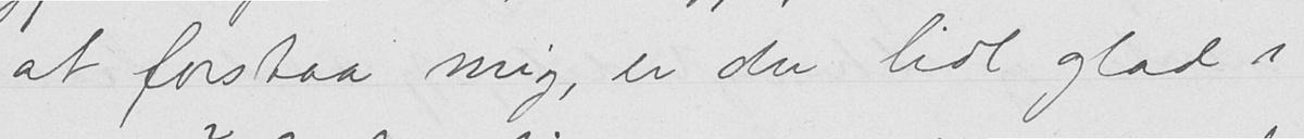at forstaa mig, er du lidt glad i
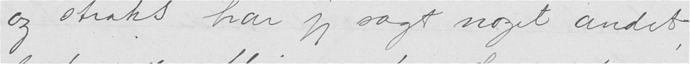og straks har jeg sagt noget andet,
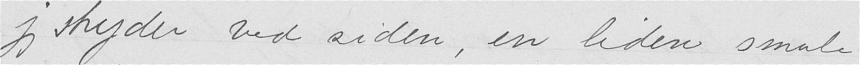jeg skyder ved siden, en liden smule
